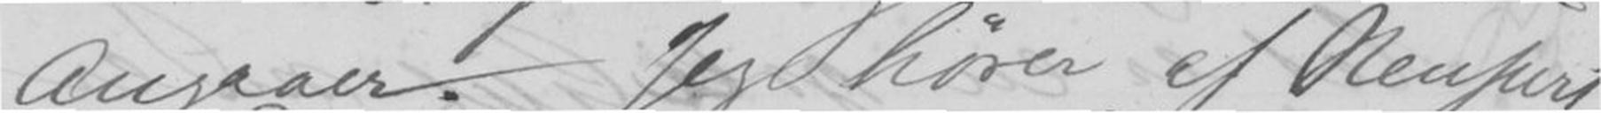Angaar. Jeg hører af Neupert
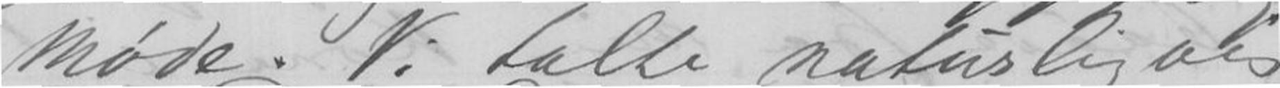Møde. Vi talte naturligvis
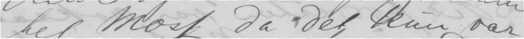til Moss da det kun var
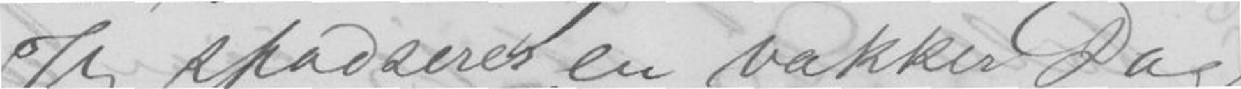Jeg spadsered en vakker Dag
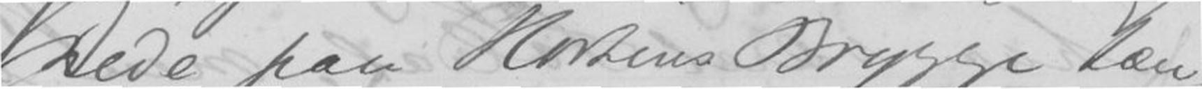nede paa Hortens Brygge tæn-
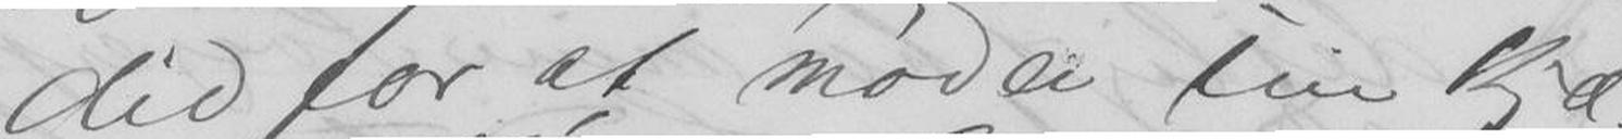did for at møde sin Kjæ-
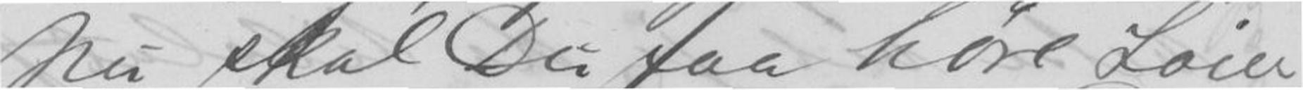Nu skal Du faa høre Loier.
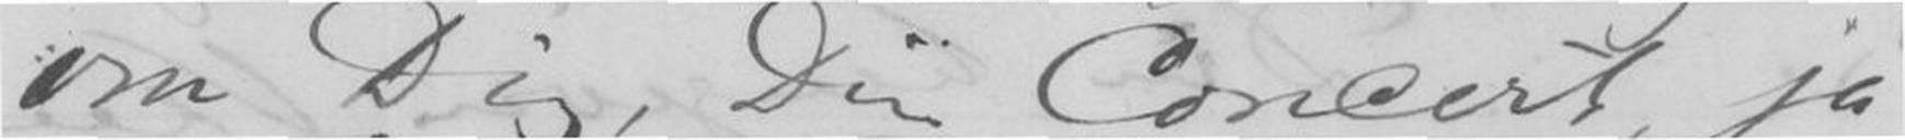om Dig, Din Concert, ja
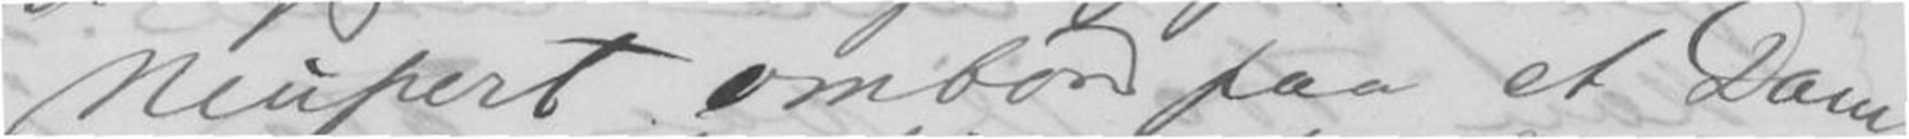Neupert ombord paa et Damp-
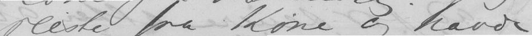reiste fra Kone og havde
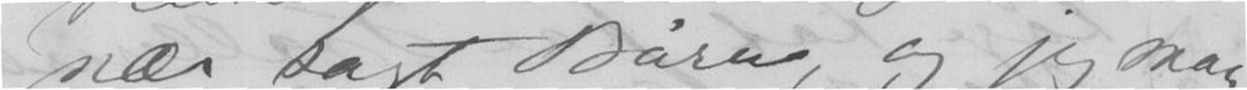nær sagt Børn, og jeg maa
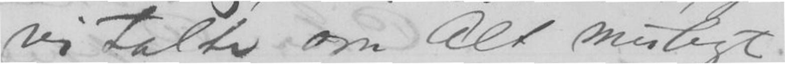vi talte om Alt muligt
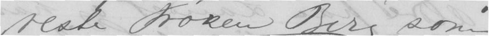reste Frøken Berg som
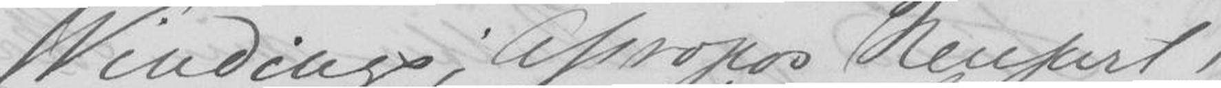Windings, Apropos Neupert!
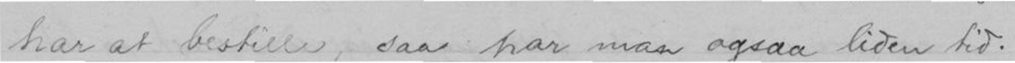har at bestille, saa har man ogsaa liden tid
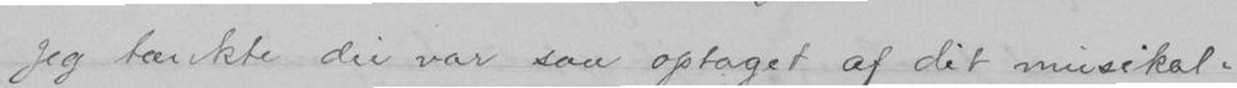Jeg tænkte du var saa optaget af dit musikalske
